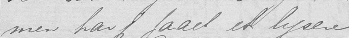men har jeg faaet et lysere
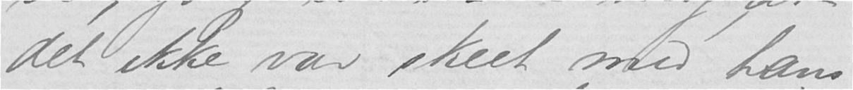det ikke var skeet med hans
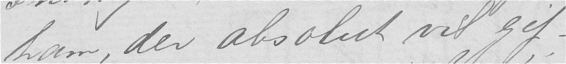ham, der absolut vil gif-
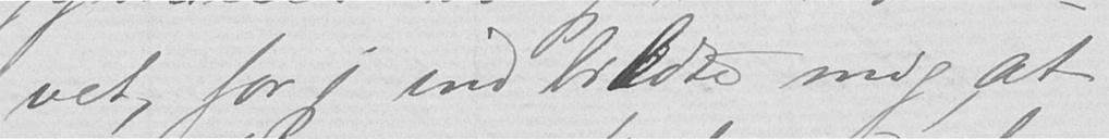vet, for jeg indbildte mig, at
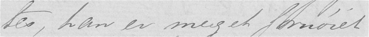tes, han er meget fornøiet
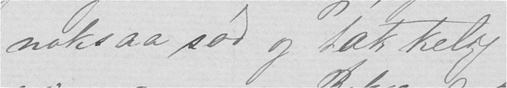noksaa sød og tækkelig
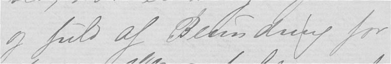og fuld af Beundring for
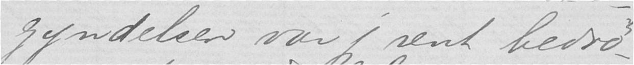gyndelsen var jeg rent bedrø-
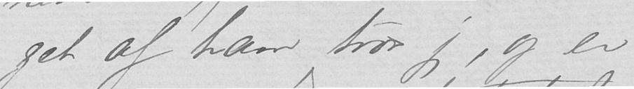get af ham tror jeg, og er
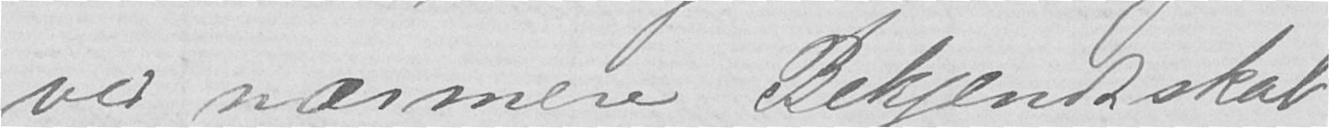ved nærmere Bekjendtskab.
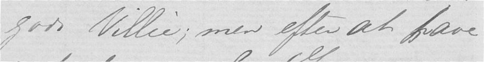gode Villie; men efter at have
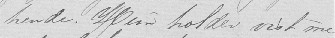hende. Hun holder vist me-
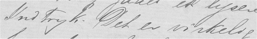Indtryk. Det er virkelig
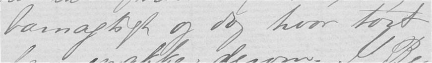barnagtigt og dog hvor tørt
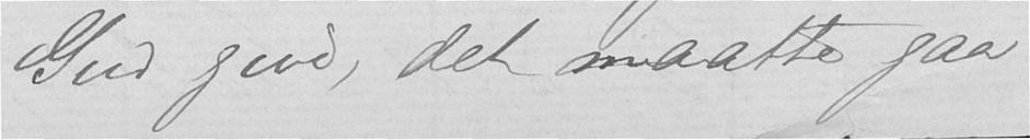Gud give, det maatte gaa
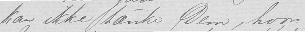kan ikke tænke Dem, hvor
